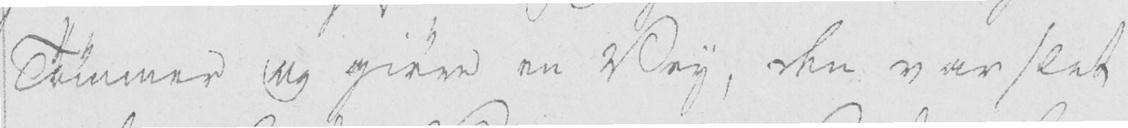Tømmer og giøre en Vey, den var slet
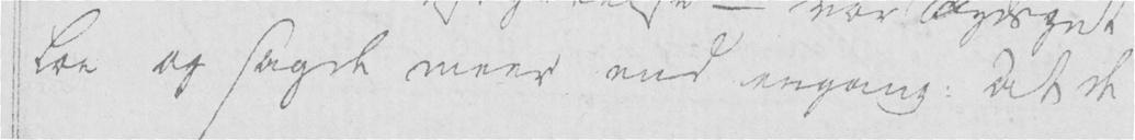loe og sagde meer end engang: at de
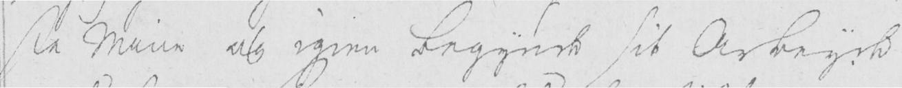ste Mine og igien begynde sit Arbeyde
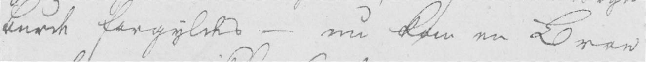burde forgyldes - nu kom en Broe
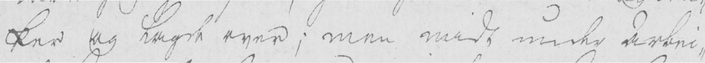ker og lagde over; men midt under Arbei-
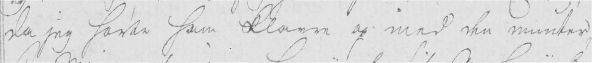da jeg hørte ham klavre op med den muntre-
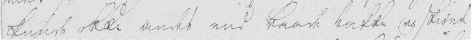kunde ikke andet end baade takke og skiænde
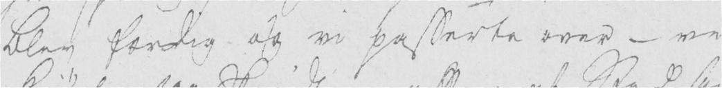blev færdig og vi passerte over - vi
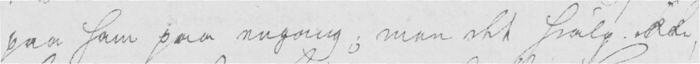paa ham paa engang; men det hialp ikke,
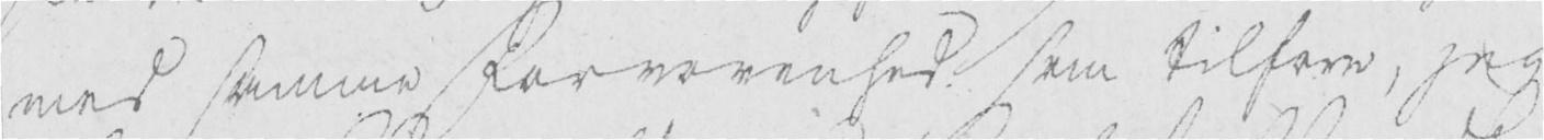med samme forvovenhed som tilforn, jeg
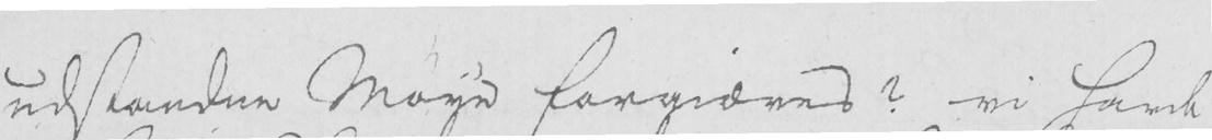udstandne Møye forgiæves? vi havde
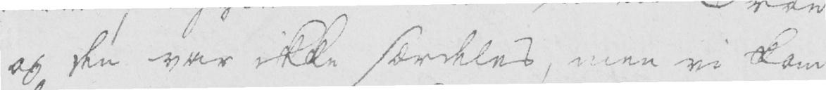og den var ikke særdeles, men vi kom
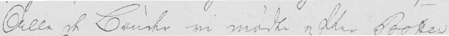Alle de Bønder vi mødte efter Botten
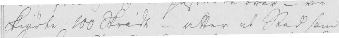kiørte 100 Skridt - atter et Sted som
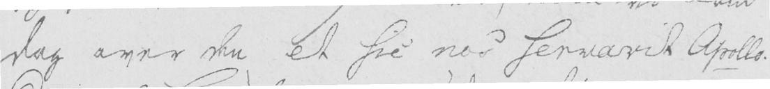dog over den et sic nos servavit Apollo.
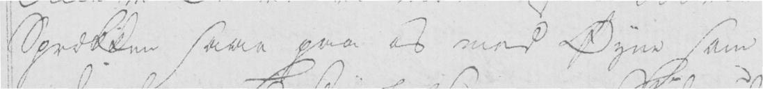Sprækken saae paa os med Øyne som
